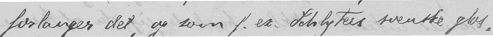forlanger det, og som f. ex. Schlyters svenske glos-
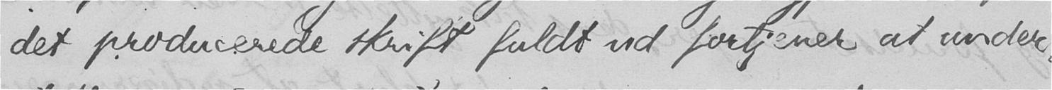det producerede skrift fuldt ud fortjener at under-
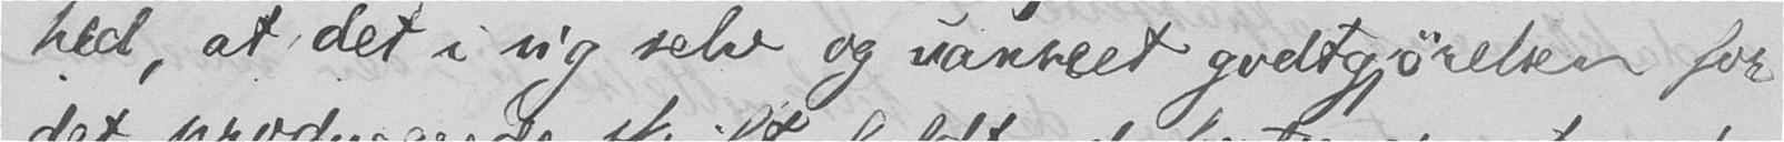hed, at det i sig selv og uanseet godtgjørelsen for
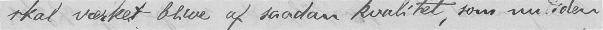skal værket blive af saadan kvalitet, som nutiden
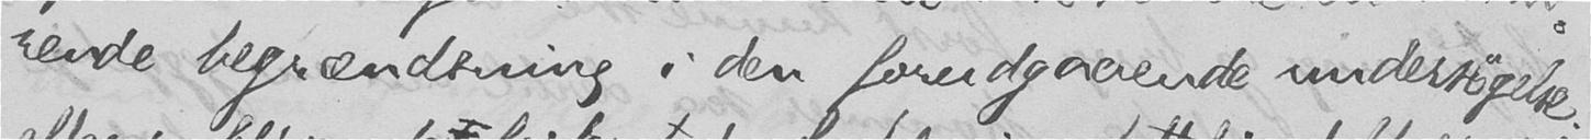rende begrændsning i den forudgaaende undersøgelse;
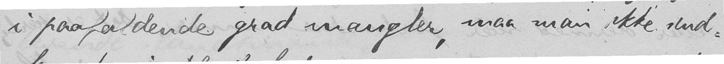i paafaldende grad mangler, maa man ikke ind-
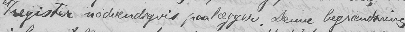register nødvendigvis paalægger. Denne begrændsning
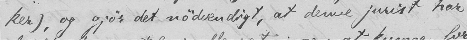ker), og gjør det nødvendigt, at denne jurist har
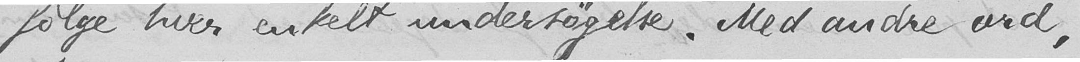følge hver enkelt undersøgelse. Med andre ord,
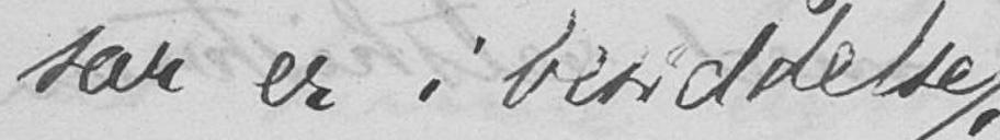sar er i besiddelse
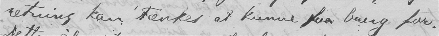retning kan tænkes at kunne faa brug for.
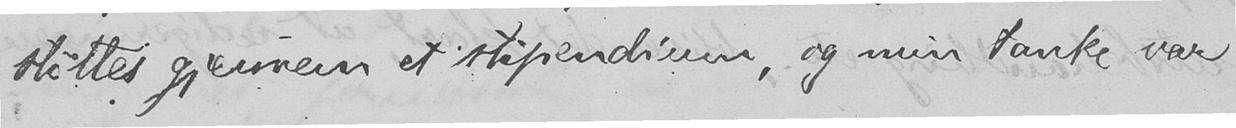støttes gjennem et stipendium, og min tanke var
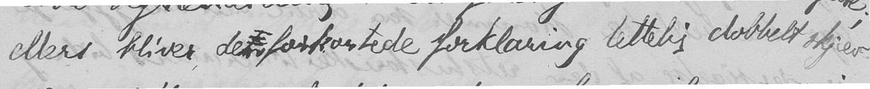ellers bliver den forkortede forklaring lettelig dobbelt skjev.
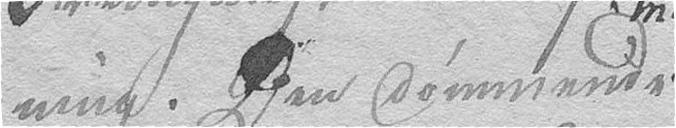ning. Den dømmende
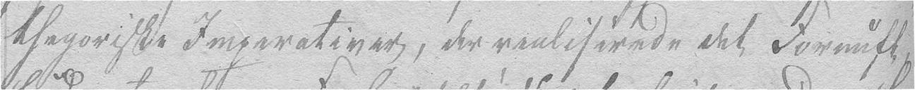thegoriske Imperativer, der realiserede det Fornuft-
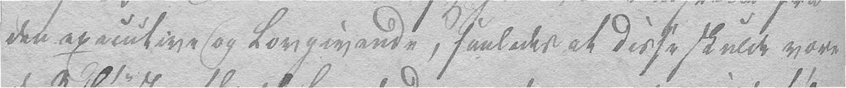den executive og Lovgivende, saaledes at disse skulde være
